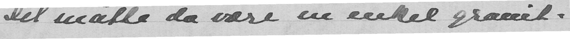Det måtte da være en enkel granit--
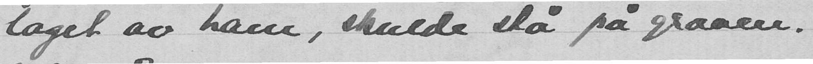laget av ham, skulde stå på graven.
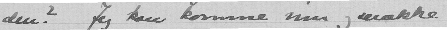den? Jeg kan komme inn og snakke
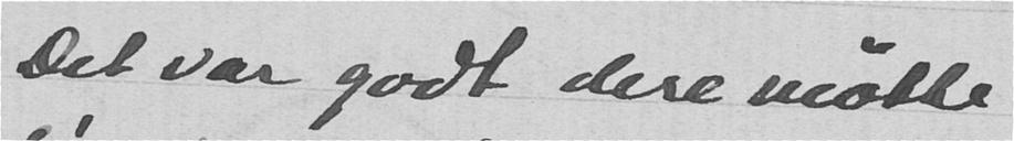Det var godt dere møtte
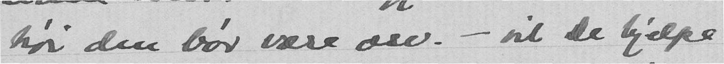høi den bør være osv. - vil De hjelpe
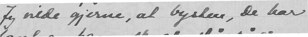Jeg vilde gjerne, at bysten, De har
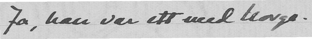Ja, han var ett med Norge.
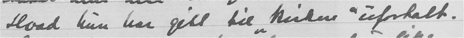Hvad hun har gitt til "kirken" ufortalt.
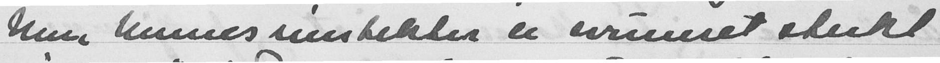Men hennes inntekter er svunnet sterkt
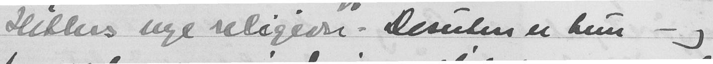Hitlers nye religion. Dessuten er hun - og
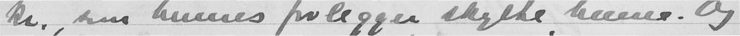kr., som hennes forlegger skylte henne. Og
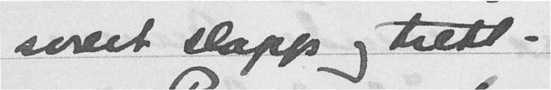svært slapp og trett -
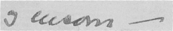og ensom -
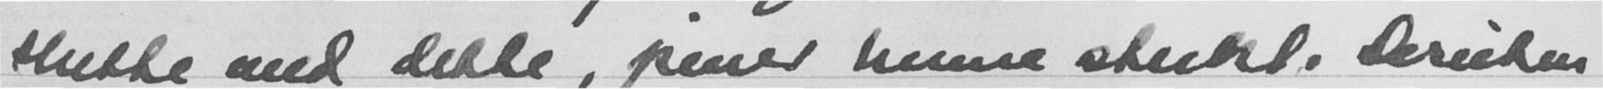slutte med dette, piner henne stort. Dessuten
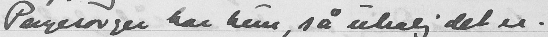Pengesorger har hun, så utrolig det er.
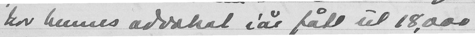har hennes advokat iår fått ut 18,000
